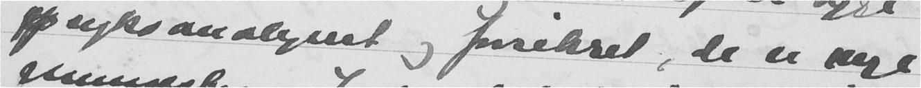psykoanalysert og forsikret, de er nye
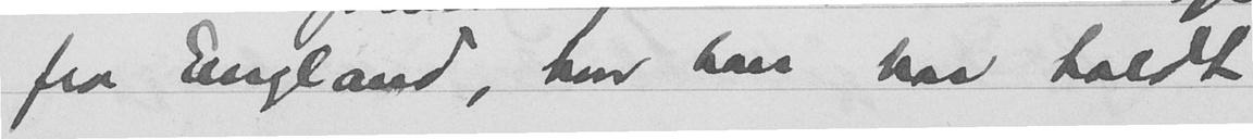fra England, hvor han har holdt
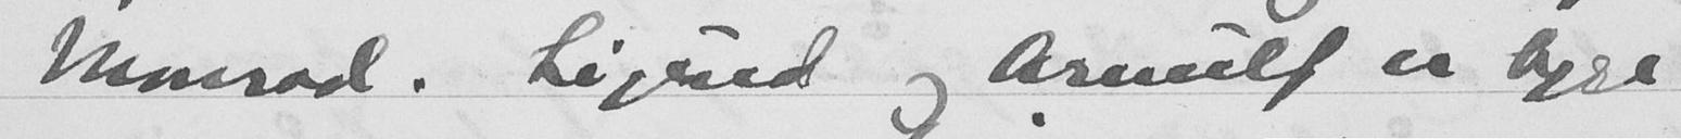Monrad. Sigurd og Arnulf er begge
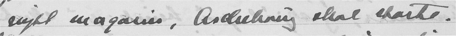nytt magasin, Asdrebang skal starte.
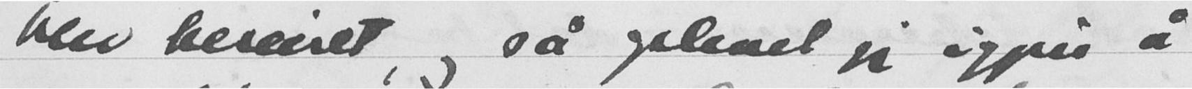blev beseiret, og så oplevet jeg igjen å
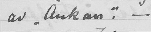av "Ankan" -
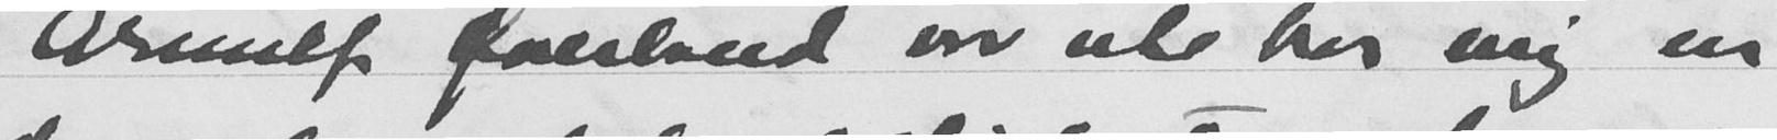Arnulf Øverland var ute hos mig en
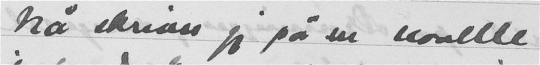Nå skriver jeg på en novelle
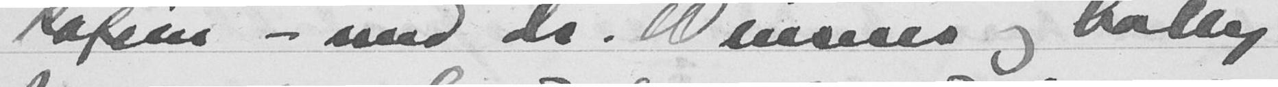kaféen og med dr. Weismes og Kally
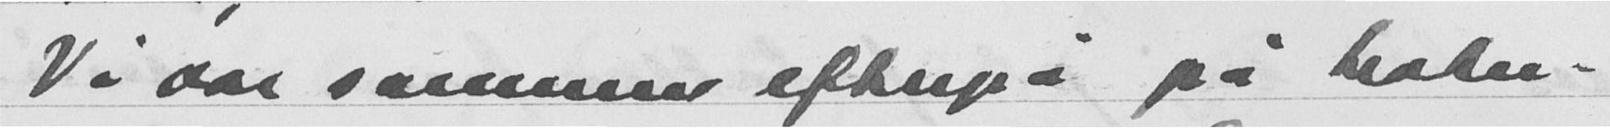Vi var sammen efterpå på teater-
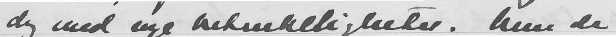dag med nye betenkeligheter. Men de
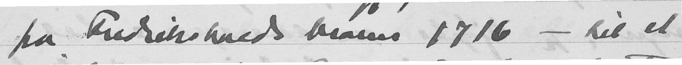fra Fredrikstads brann 1716 - til et
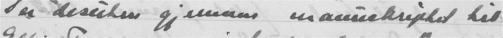Ser desuten gjennom manuskriptet til
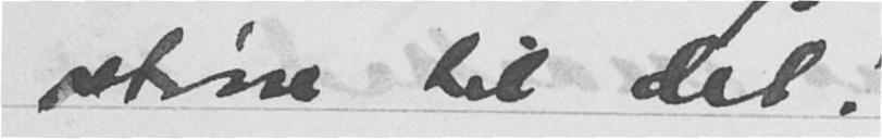skrive til det!
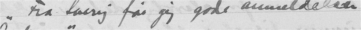Fra Sverige får jeg gode anmeldelser
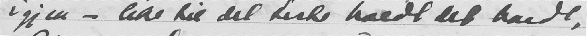igjen - like til det siste holdt det hardt.
